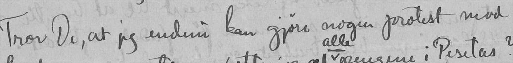Tror De, at jeg endnu kan gjøre nogen protest mod
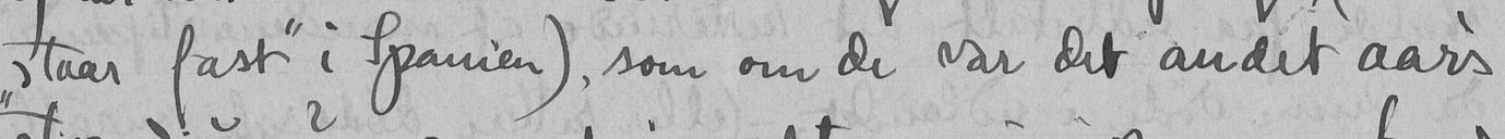"staar fast" i Spanien), som om de var det andet aars
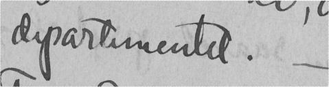departementet. -
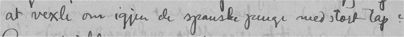at vexle om igjen de spanske penge med stort tap?
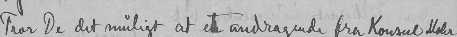Tror De det muligt at et andragende fra Konsul Mohr
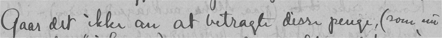Gaar det ikke an at betragte disse penge, (som nu
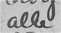alle
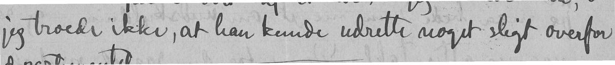jeg troede ikke, at han kunde udrette noget sligt overfor
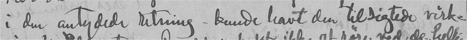i den antydede retning - kunde havt den tilsigtede virk-
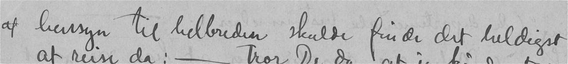af hensyn til helbreden skulde finde det heldigst
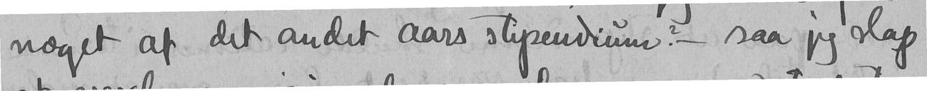noget af det andet aars stipendium? - saa jeg slap
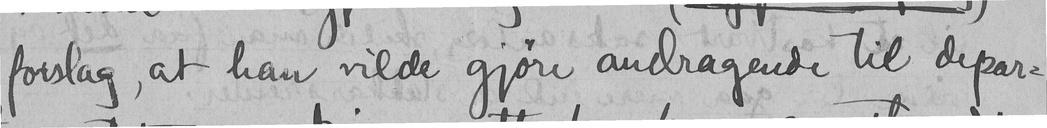forslag, at han vilde gjøre andragende til depar-
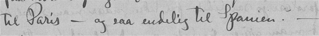til Paris - og saa endelig til Spanien? -
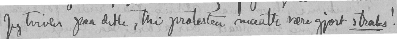Jeg tvivler paa dette, thi protesten maatte være gjort straks!
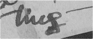ting -
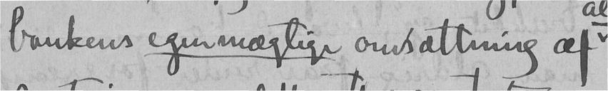bankens egenmægtige omsættning af
